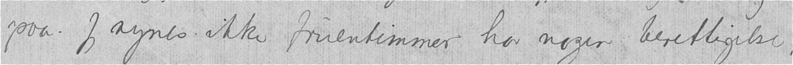paa. Jeg synes ikke fruentimmer har nogen berettigelse,
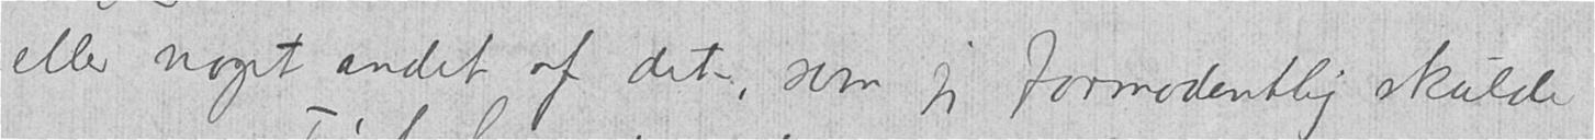eller noget andet af det, som jeg formodentlig skulde
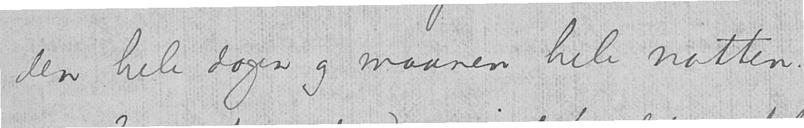den hele dagen og maanen hele natten.
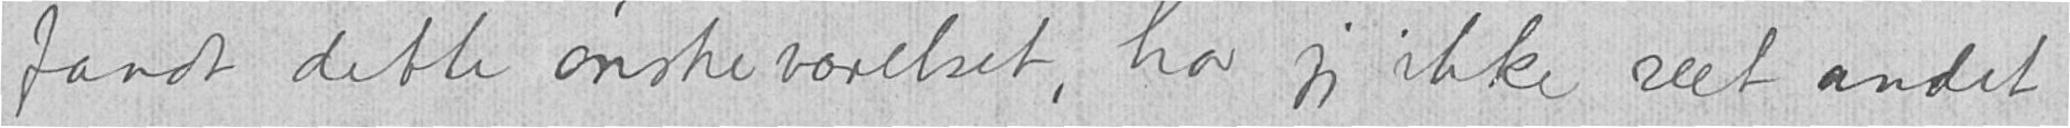fandt dette ønskeværelset, har jeg ikke seet andet
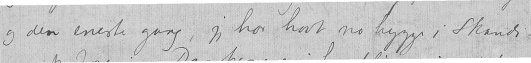og den eneste gang, jeg har havt no hygge i Skandi-
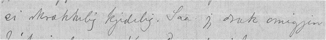si skrækkelig kjedelig. Saa jeg drak omigjen
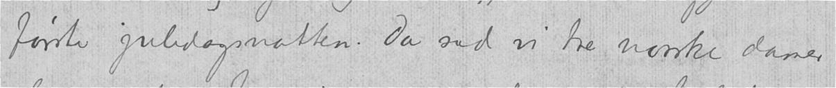første juledagsnatten. Da sad vi tre norske damer
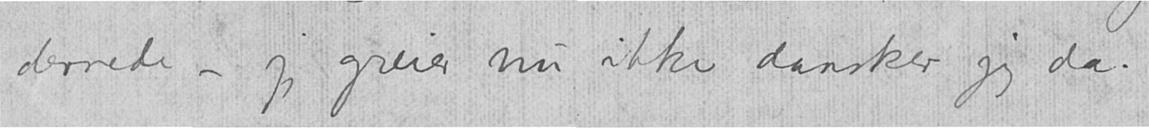dernede — jeg greier nu ikke dansker jeg da.
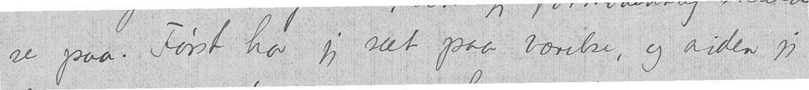se paa. Først har jeg seet paa værelse, og siden jeg
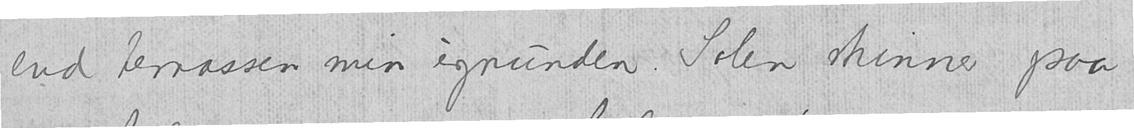end terrassen min igrunden. Solen skinner paa
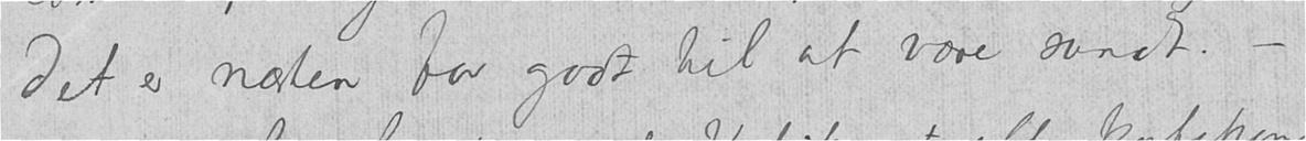Det er næsten for godt til at være sandt. -
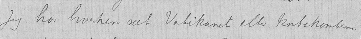Jeg har hverken set Vatikanet eller katakomberne
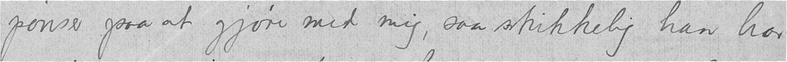pønser paa at gjøre med mig, saa skikkelig han har
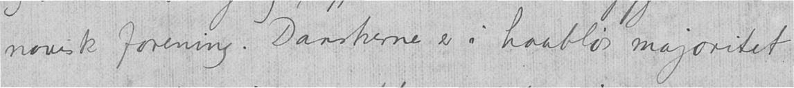navisk forening. Danskerne er i haabløs majoritet
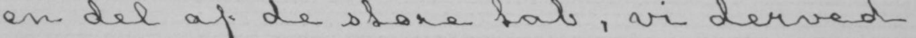en del af de store tab, vi derved
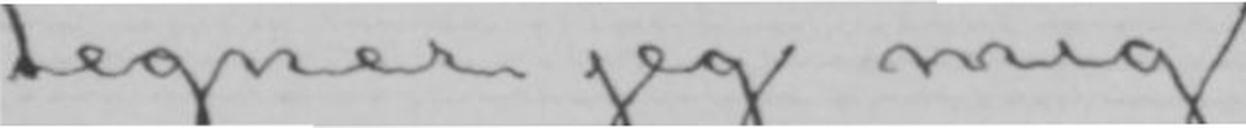tegner jeg mig
